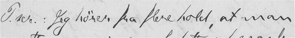P. scr.: Jeg hører fra flere hold, at man
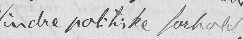indre politiske forhold,
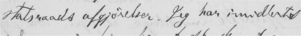statsraads afgjørelser. Jeg har imidlertid
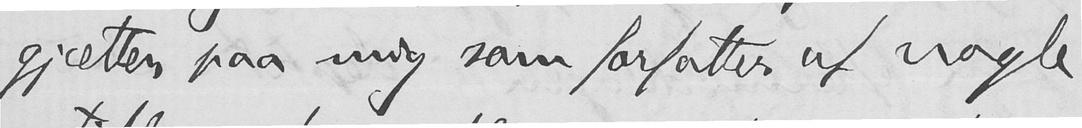gjætter paa mig som forfatter af nogle
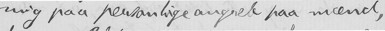mig paa personlige angreb paa mænd,
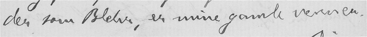der som Blehr, er mine gamle venner.
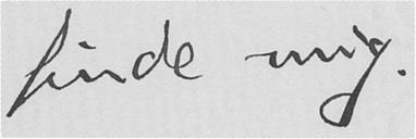finde mig.
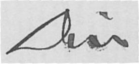Din
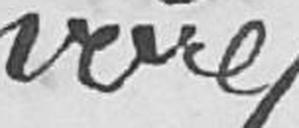vore
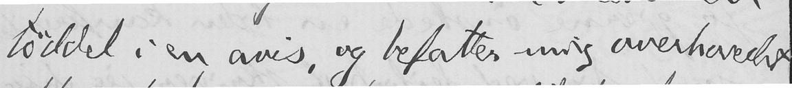tøddel i en avis, og befatter mig overhovedet
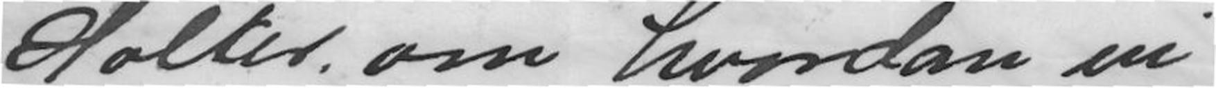Holter. om hvordan vi
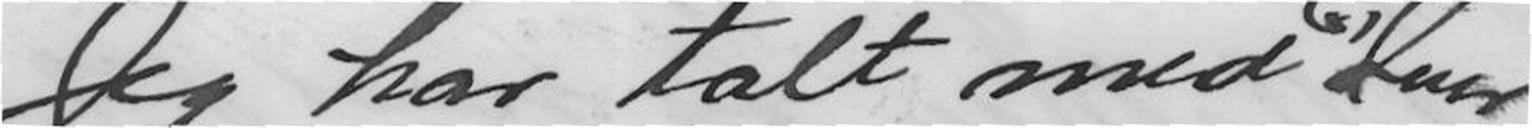Jeg har talt med Iver
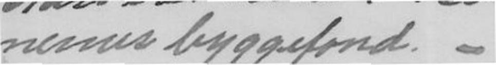nernes byggefornd. -
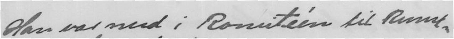Han var med i komitèen til kunst-
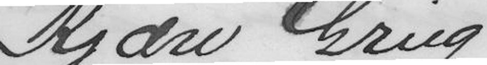Kjære Grieg!
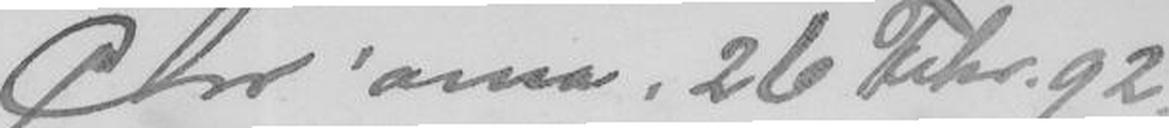Chr'ania. 26 Febr. 92
